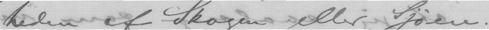heden af Skogen eller Sjøen.
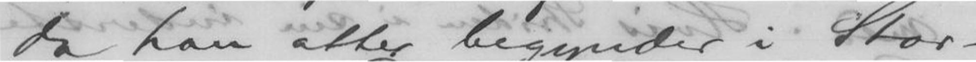da han atter begynder i Stor-
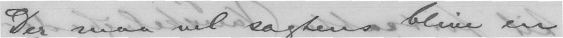Der maa vel sagtens blive en
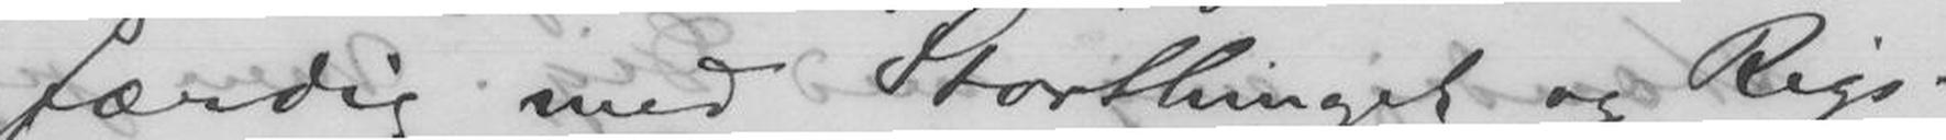færdig med Storthinget og Rigs-
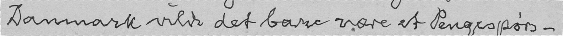Danmark vilde det bare være et Pengespørs-
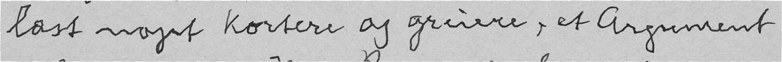læst noget kortere og greiere, et Argument
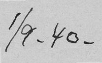1/9. 40.
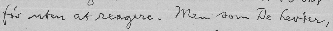før uten at reagere. Men som De hevder,
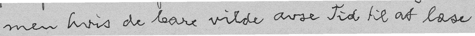men hvis de bare vilde avse Tid til at læse
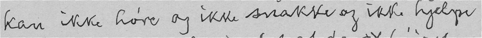kan ikke høre og ikke snakke og ikke hjelpe
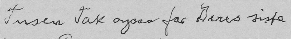Tusen Tak ogsaa for Deres siste
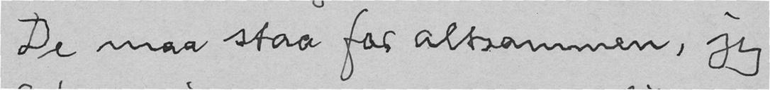De maa staa for altsammen, jeg
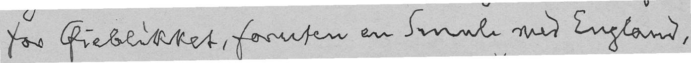for Øieblikket, foruten en Smule med England,
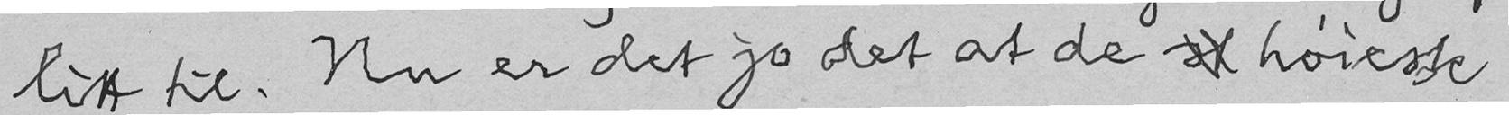litt til. Nu er det jo det at de sl høieste
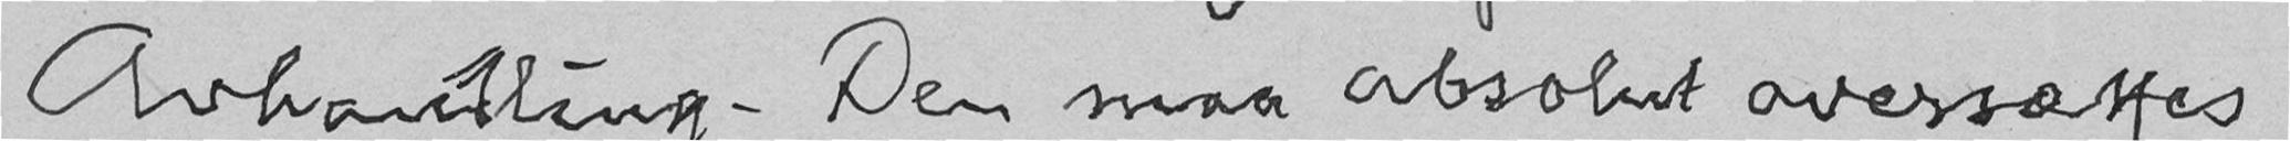Avhandling. Den maa absolut oversættes
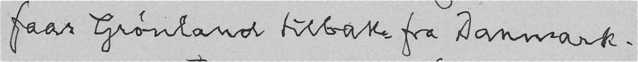faar Grønland tilbake fra Danmark.
